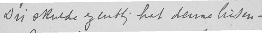Du skulde egentlig hat denne hilsen-
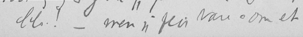Chr.!  - men jeg fløi bare som et
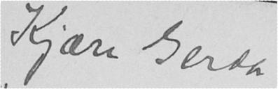Kjære Gerda
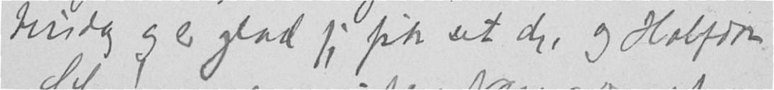tirsdag og er glad jeg fik set dig og Halfdan
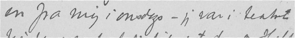en fra mig i onsdags – jeg var i teatret
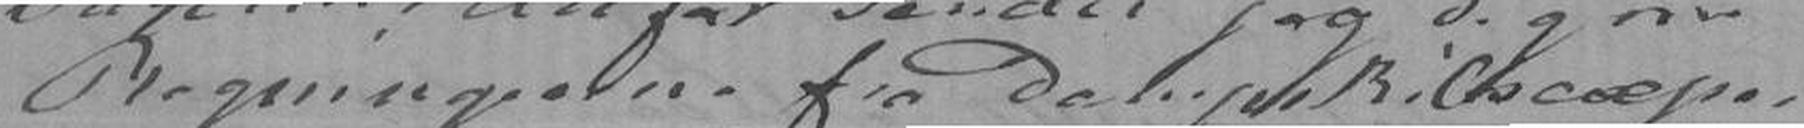Regningerne fra Dampskibexpe-
